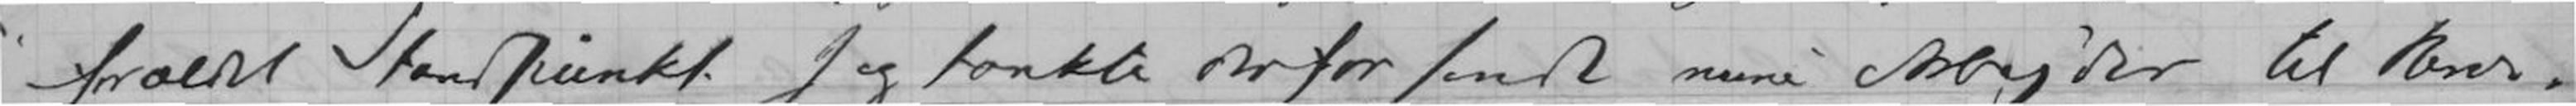forældet Standpunkt. Jeg tænkte derfor sendt mine Arbejder til Henr.
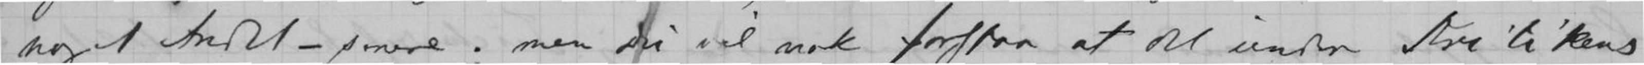Noget Andet - senere; men du vil nok forstaa at det under Kritikens
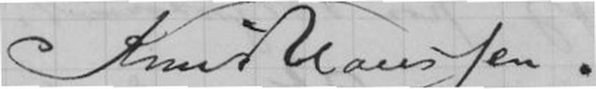Knud Claussen.
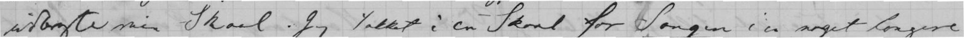udbragte min Skaal. Jeg takket i en Skaal for Sangen i en noget lengre
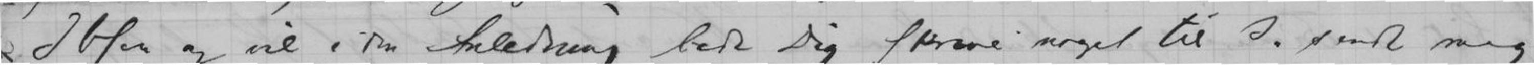Ibsen og vil i den Anledning bede Dig skrive noget til I. sende mig
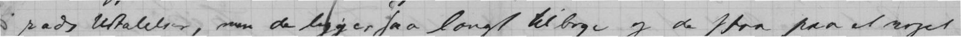rads Udtalelser, men de ligger saa langt tilbage og da staa paa et noget
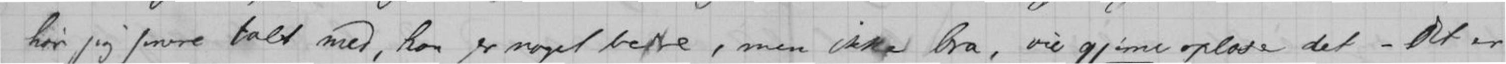har jeg senere talt med, han er noget bedre, men ikke bra, vil gjerne oplese det - Det er
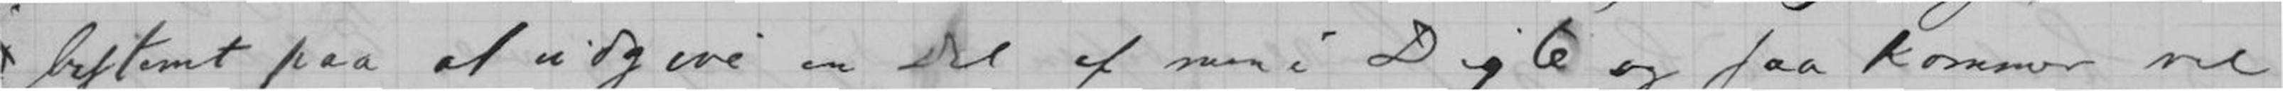bestemt paa at udgive en Del af mine Digte og saa kommer vel
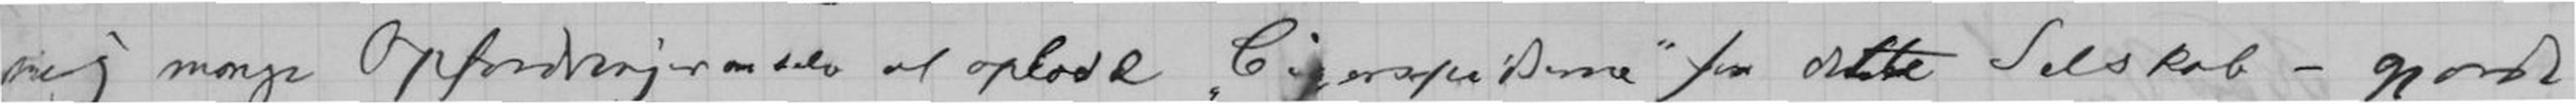mig mange Opfordringer at opleve. "Cigarspidserne" for dette Selskab - gjorde
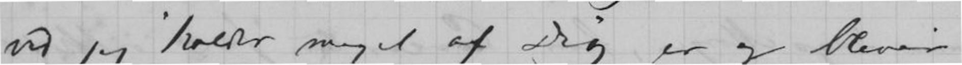ved jeg holder af Dig er og bliver
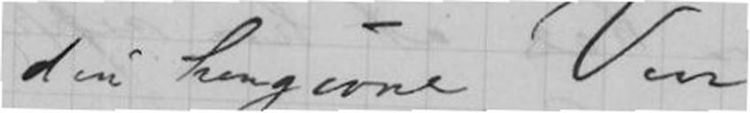Din hengivne Ven
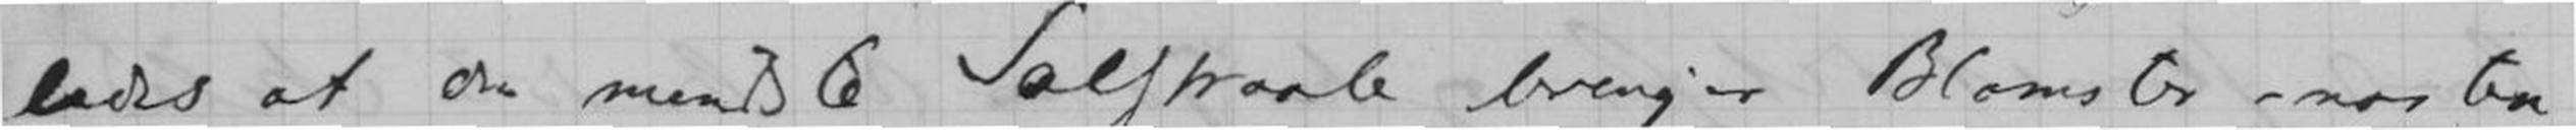ledes at du mindste Solstraale bringer Blomster næsten
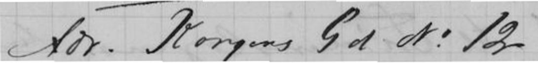Adr. Kongens Gd No 12
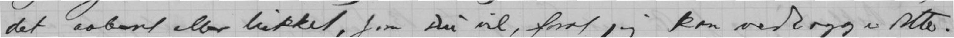det aabent eller lukket, som Du vil, forat jeg kan vedlegge dette.
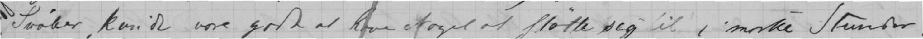Svøber, kan de være godt at have Noget at støtte seg til i mørke Stunder, det
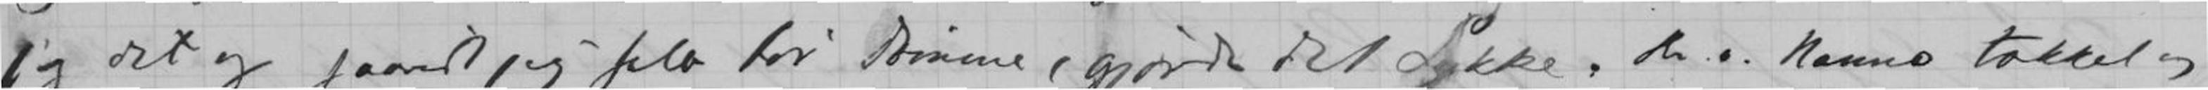sig det og saavidt jeg selv tør drømme, gjorde det Lykke. Dr. Nanno takket og
